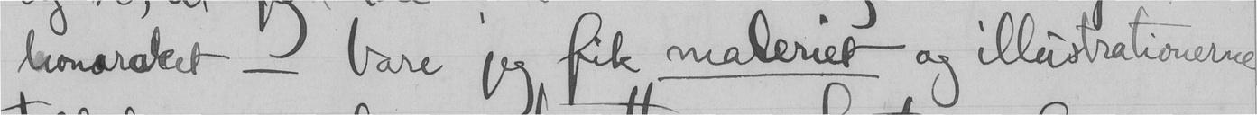honoraret - bare jeg fik maleriet og illusrationerne
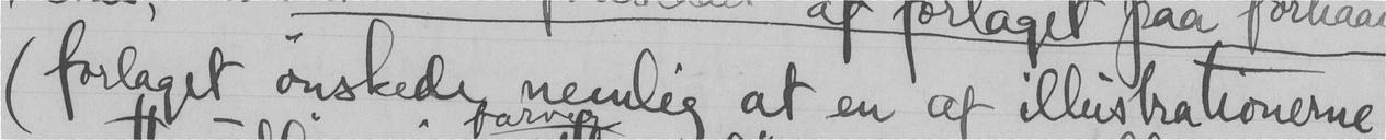(forlaaet ønskede nemlig at en af illustrationerne
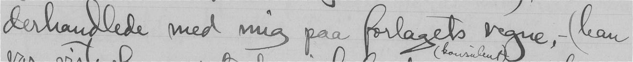derhandlede med mig paa forlagets vegne, - (han
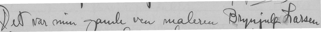Det var min gamle ven maleren Brynjulf Larsen
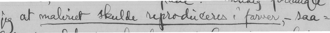jeg at maleriet skulde reproduceres i farver, - saa-
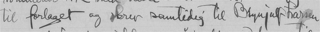til forlaget og skrev samtidig til Brynjulf Larsen
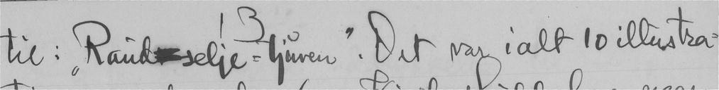til: "Raud selje tjuven". Det var ialt 10 illustra-
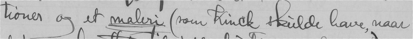tioner og et maleri (som Kinck skulde have, naar
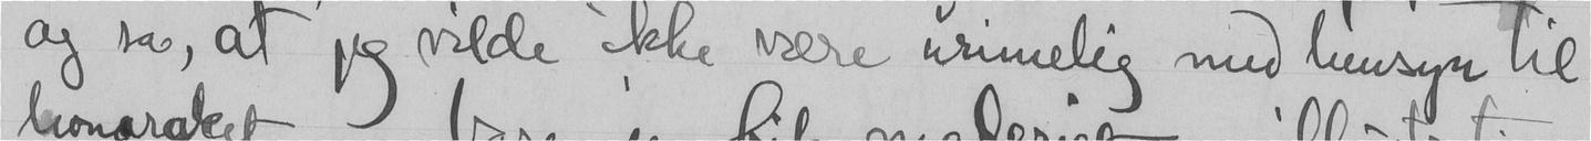og sa, at jeg vilde ikke være urimelig med hensyn til
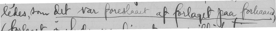ledes, som det var foreslaaet af forlaget paa forhaand
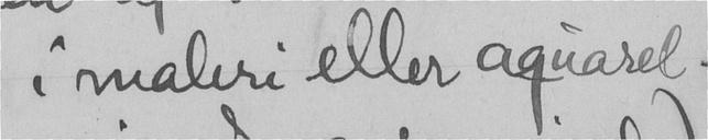i maleri eller aquarel -
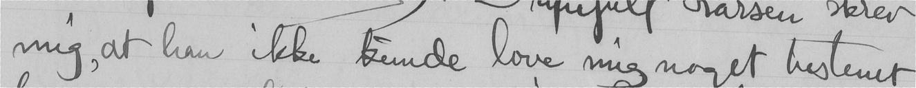mig, at han ikke kunde love mig noget bestemt
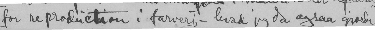for reproductson i farver, - hvad jeg da ogsaa gjorde)
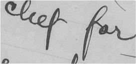chef for
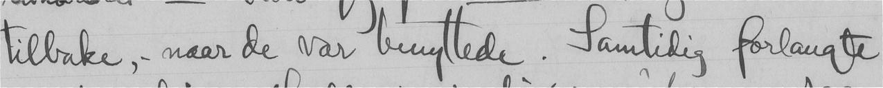tilbake, - naar de var benyttede. Samtidig forlangte
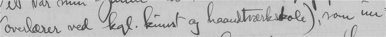(overlærer ved kgl. kunst og haandtværkskole), som un-
